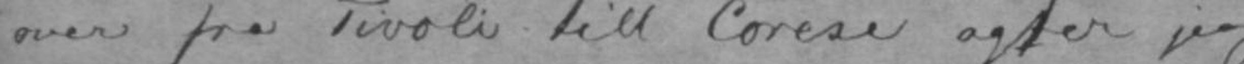over fra Tivoli till Corese agterag jeg
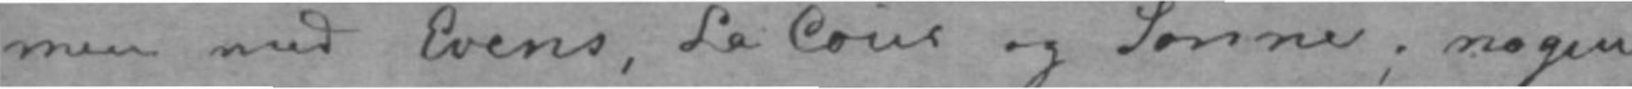men med Evens, La Cour og Sonne; nogen
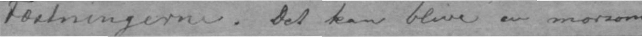Fæstningerne. Det kan blive en morsom
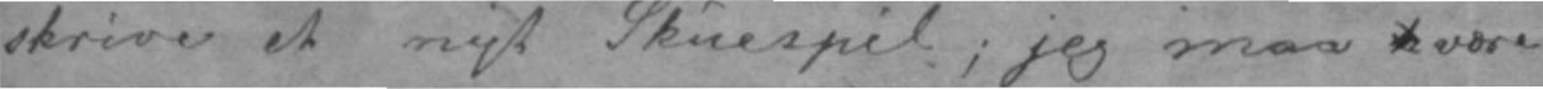skrive et nyt Skuespil; jeg maa  være
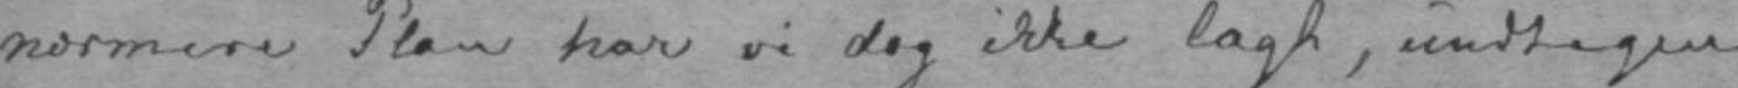nærmere Plan har vi dog ikke lagt, undtagen
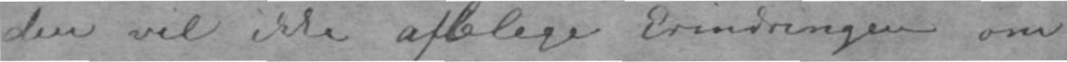den vil ikke afblege Erindringen om
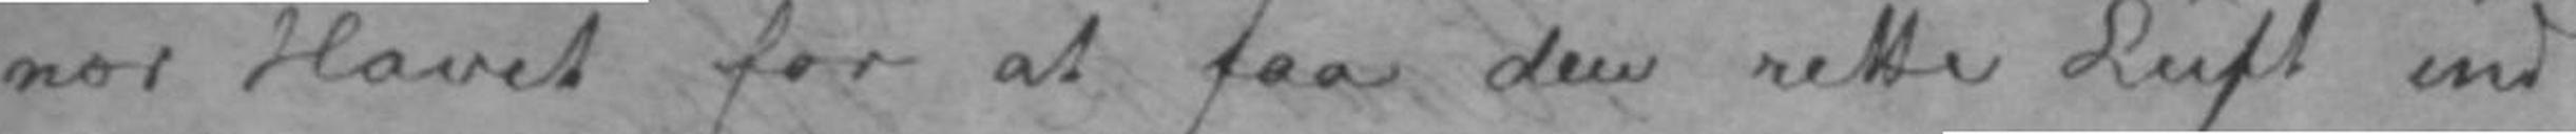nær Havet for at faa den rette Luft ind
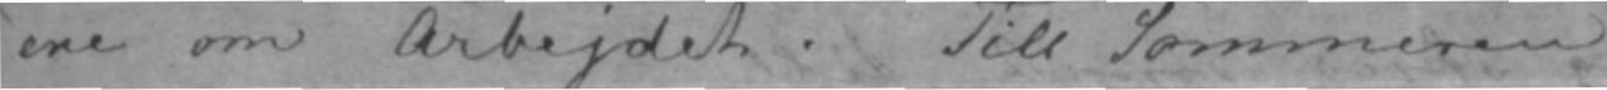ene om Arbejdet. Till Sommeren
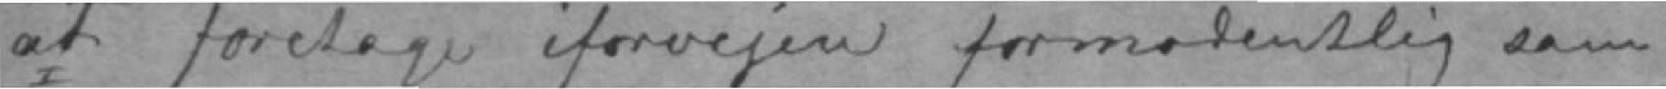at foretage iforvejen formodentlig sam-
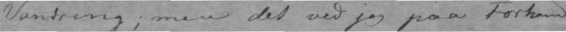Vandring; men det ved jeg paa Forhaand,
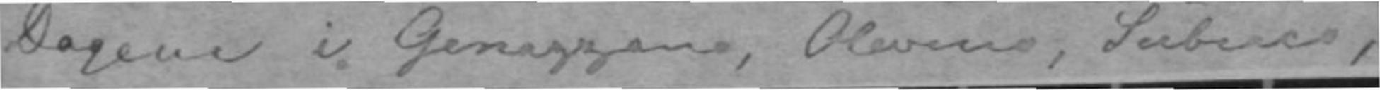Dagene i Genazzano, Olevano, Subiaco,
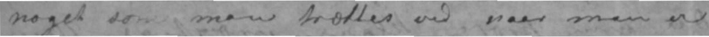noget som man trættes ved naar man er
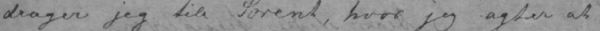drager jeg till Sorent, hvor jeg agter at
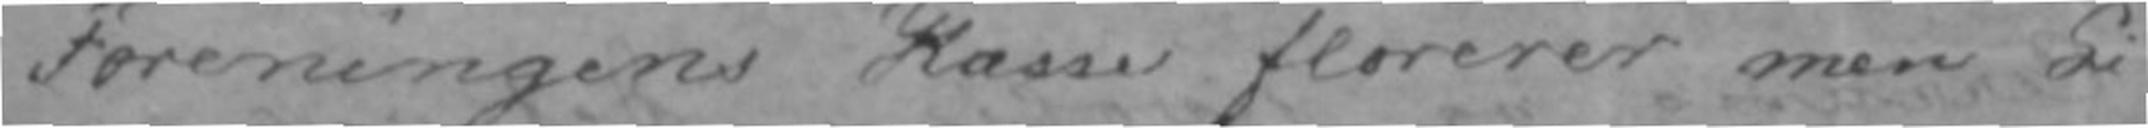Foreningens Kasse florerer men Li-
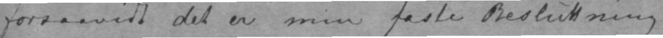forsaavidt det er min faste Besluttning
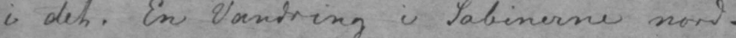i det. En Vandring i Sabinerne nord-
